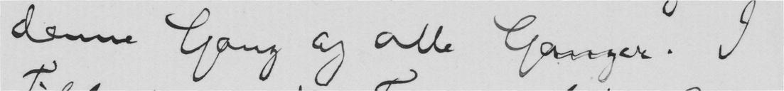denne Gang og alle Ganger. I
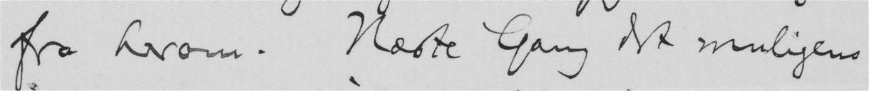fra herom. Næste Gang det muligens
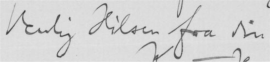Venlig Hilsen fra din
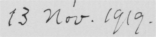13 Nov. 1919.
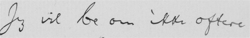Jeg vil be om ikke oftere
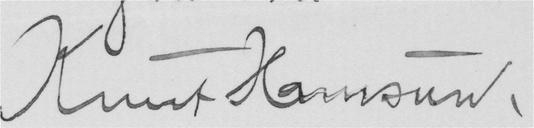Knut Hamsun.
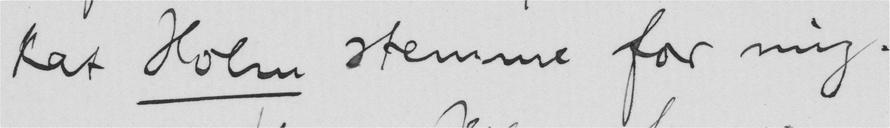kat Holm stemme for mig.
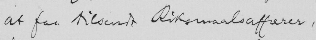at faa tilsendt Riksmaalsaffærer,
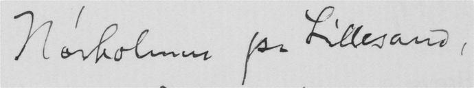Nørholm pr Lillesand,
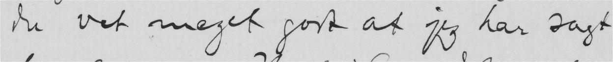du vet meget godt at jeg har sagt
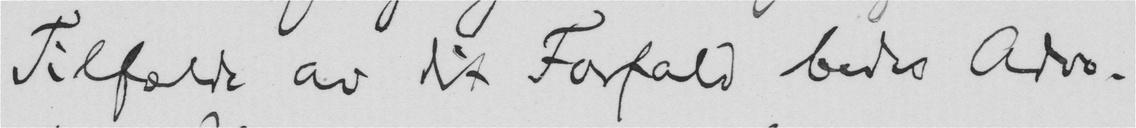Tilfælde av dit Forfald bedes Advo-
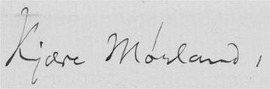Kjære Mørland,
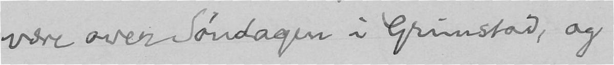være over Søndagen i Grimstad, og
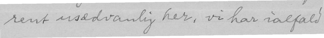rent usædvanlig her, vi har ialfald
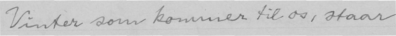Vinter som kommer til os, staar
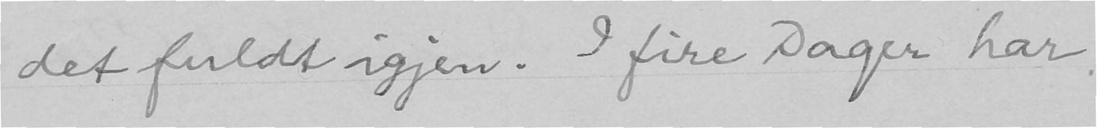det fuldt igjen. I fire Dager har
